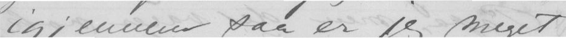igjennem saa er jeg meget
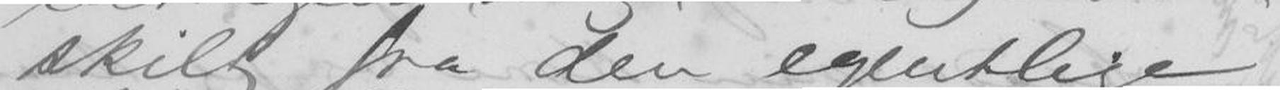skilt fra den egentlige
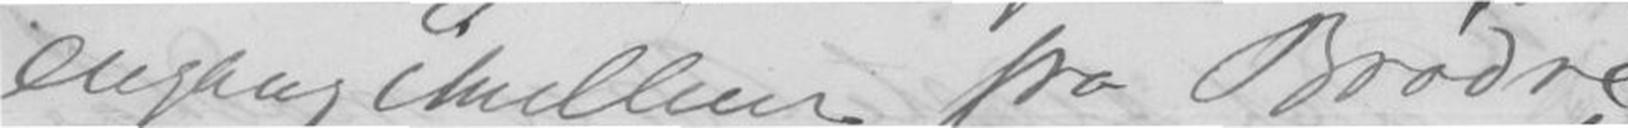engang imellem fra Brødre
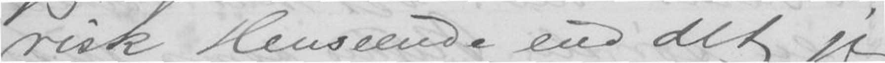risk Henseende end det jeg
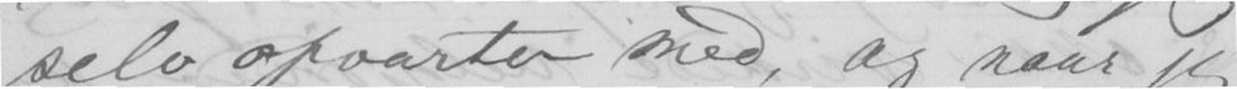selv opvarter med, og naar jeg
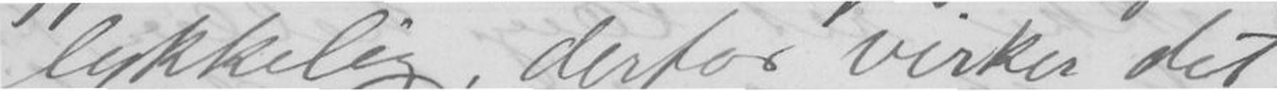lykkelig, derfor virker det
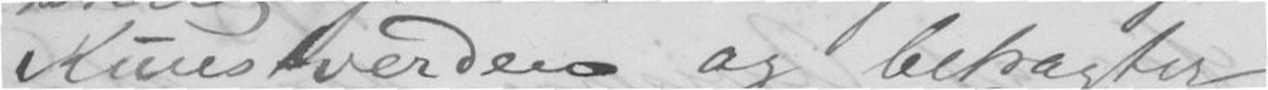Kunstverden og betragter
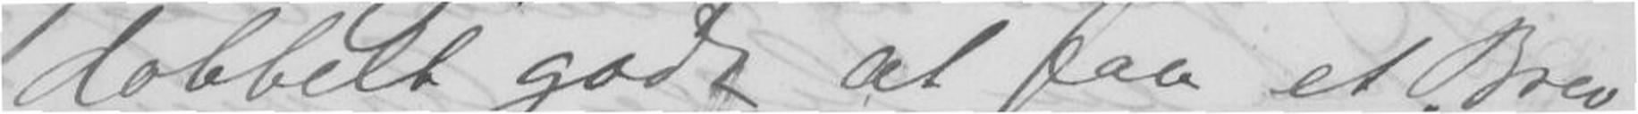dobbelt godt at faa et Brev
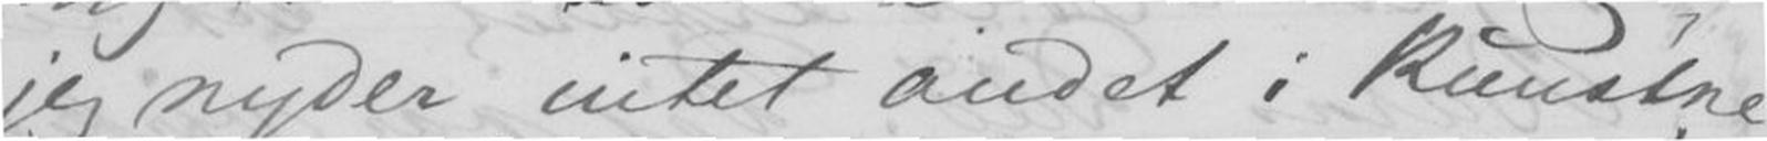jeg nyder intet andet i kunstne-
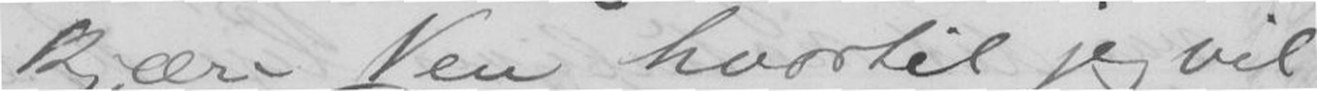kjære Ven hvortil jeg vil
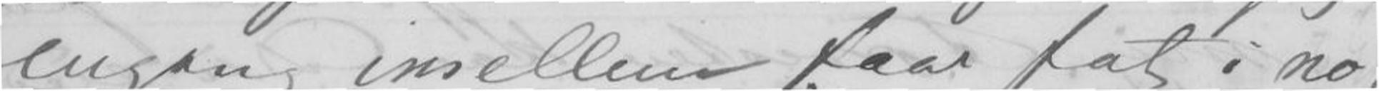engang imellem faar fat i no-
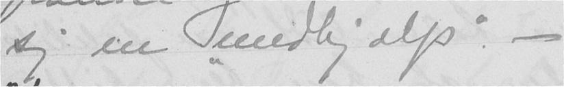sig en "medhjælp" -
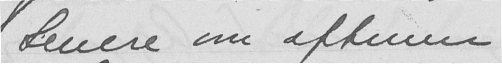Senere om aftenen
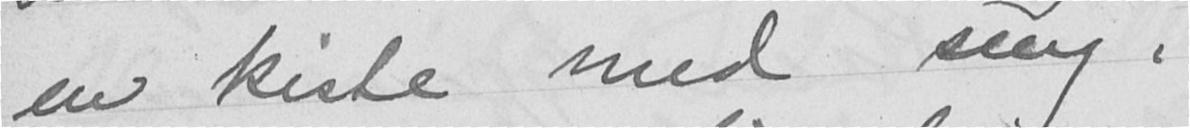en kiste med seng-
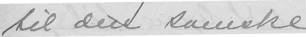til den svenske
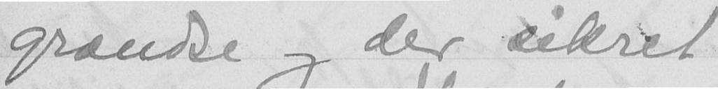grændse og der sikret
